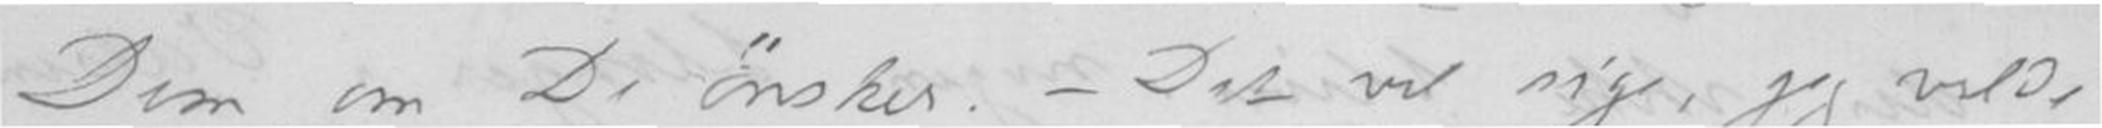Dem, om De ønsker. - Det vil sige, jeg vilde
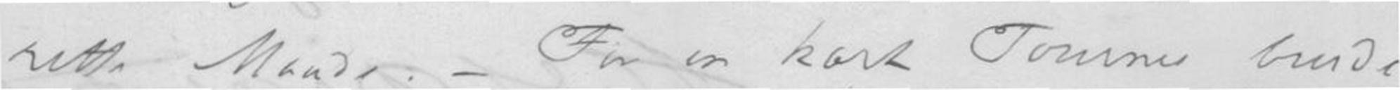rette Maade. - For en kort Tourne burde
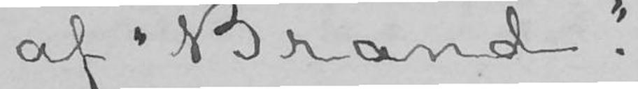af «Brand».
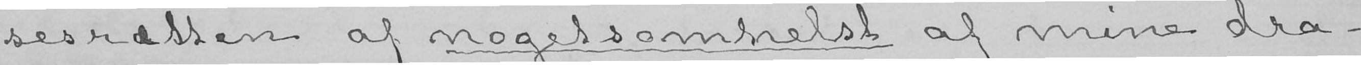sesretten af nogetsomhelst af mine dra-
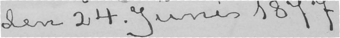den 24. Juni 1877.
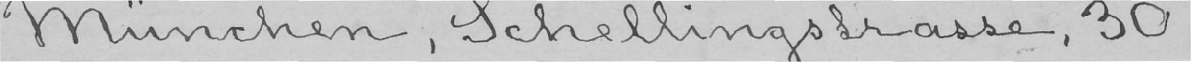München, Schellingstrasse, 30,
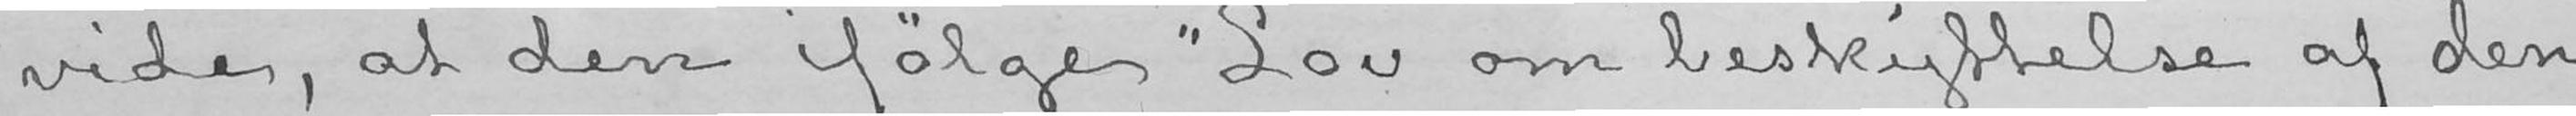vide, at den ifølge «Lov om beskyttelse af den
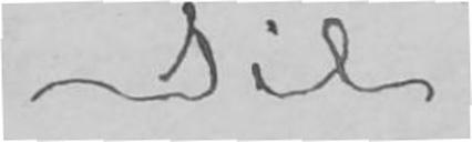Til
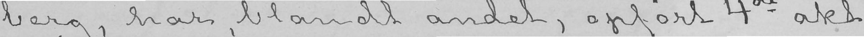berg, har, blandt andet, opført 4de akt
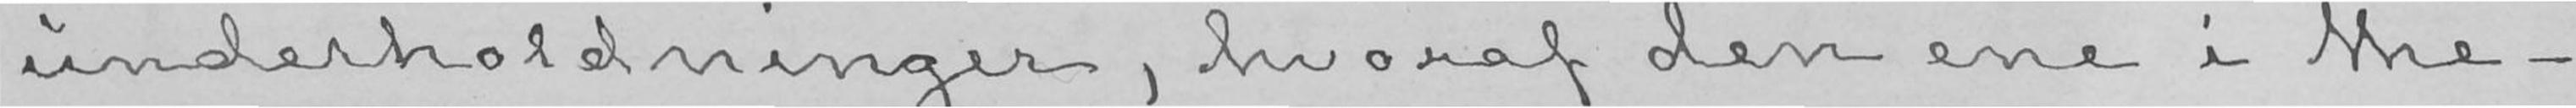underholdninger, hvoraf den ene i the-
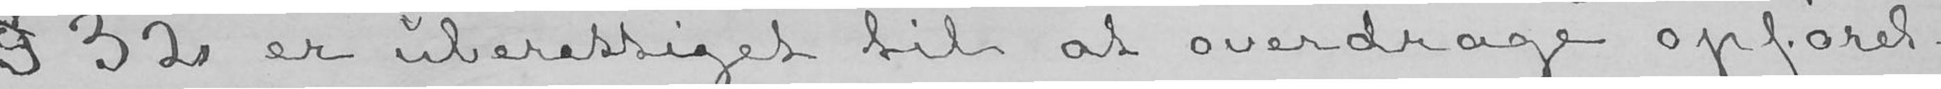§ 32 er uberettiget til at overdrage opførel-
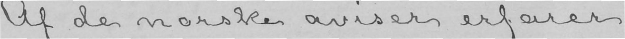Af de norske aviser erfarer
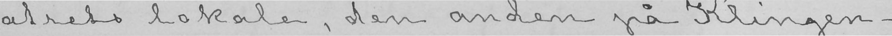atrets lokale, den anden på Klingen-
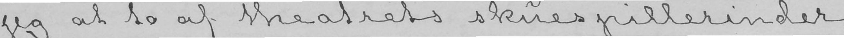jeg at to af theatrets skuespillerinder
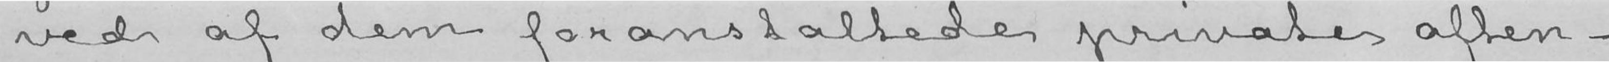ved af dem foranstaltede private aften-
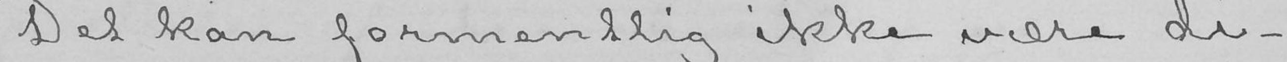Det kan formentlig ikke være di-
